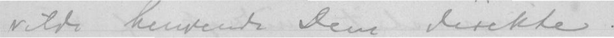vilde henvende Dem direkte.
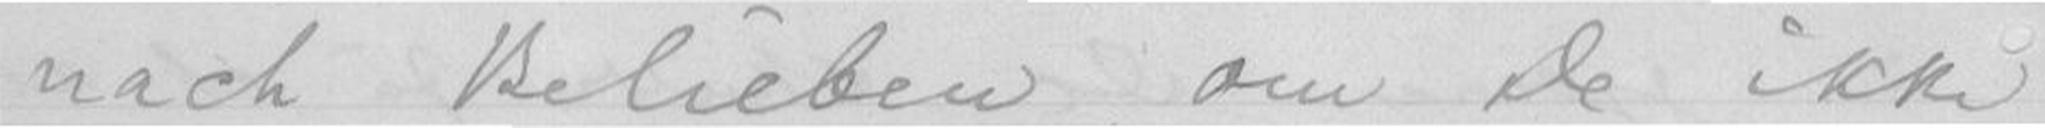nach Belieben, om De ikke
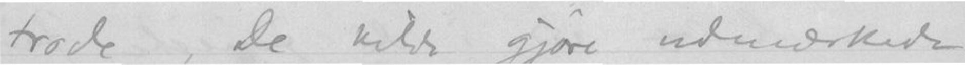trode, De vilde gjøre udmærkede
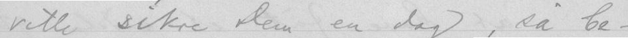ville sikre Dem en dag, så be-
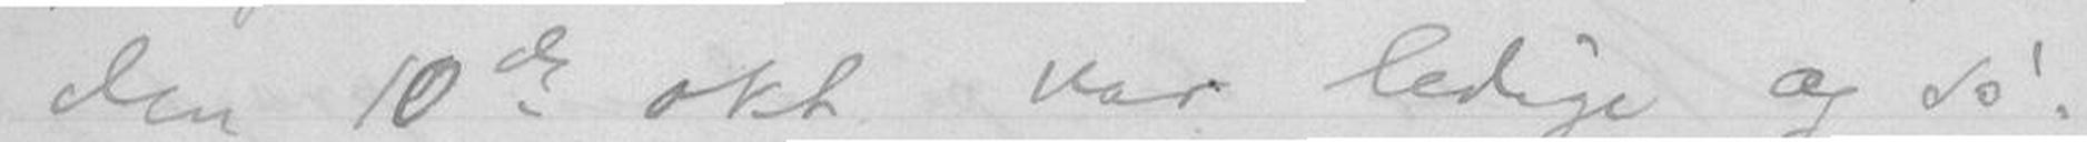den 10de okt var ledige og så-
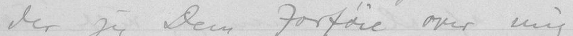der jeg Dem forføie over mig
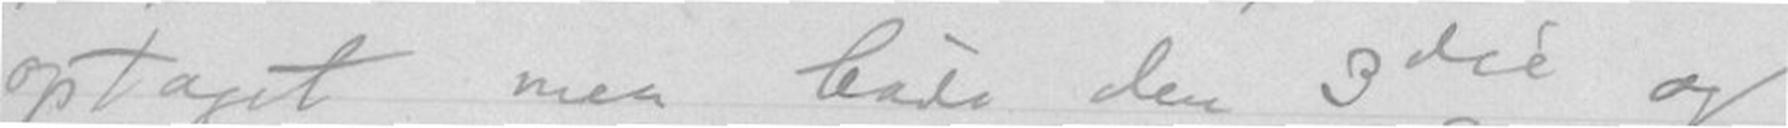optaget men både den 3die og
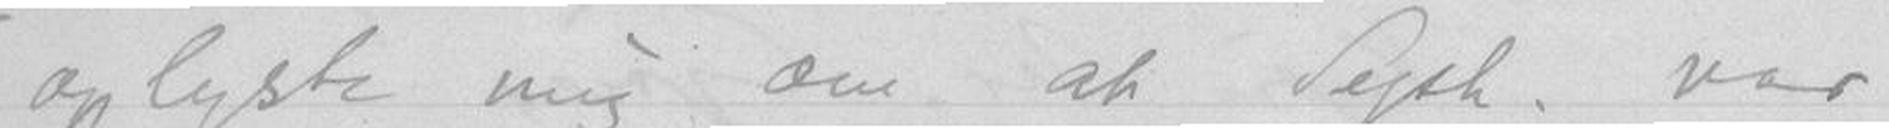oplyste mig om at Septb. var
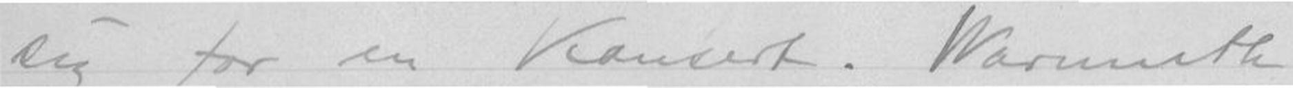sig for en konsert. Warmuth
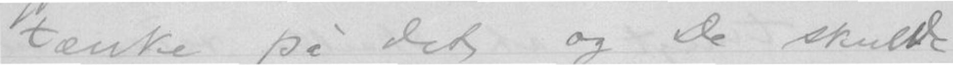tænke på det og De skulde
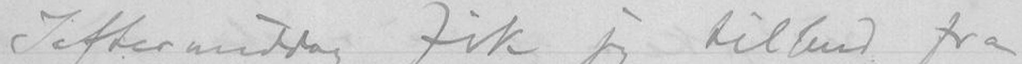Ieftermiddag fik jeg tilbud fra
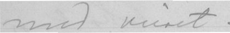med veiret.
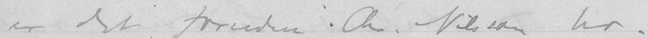er det foruden Chr. Nilsson hr.
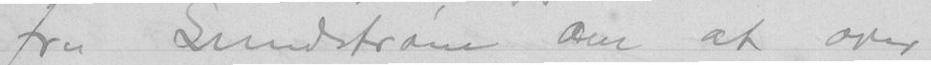fru Sundstrøm om at over
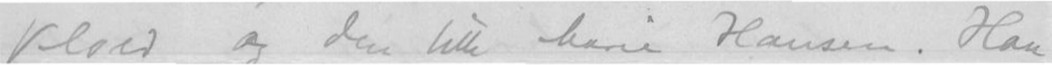Kloed og den lille Marie Hansen. Han
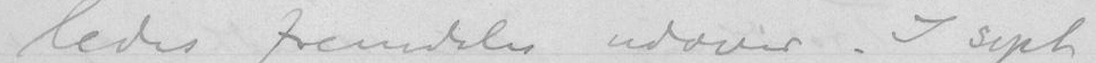ledes fremdeles udover. I sept
